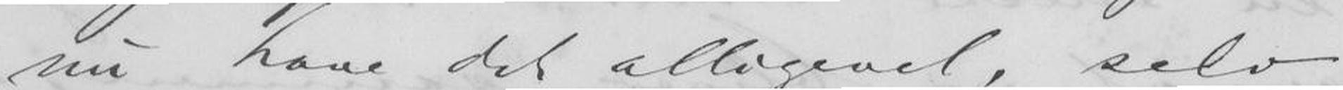nu have det alligevel, selv
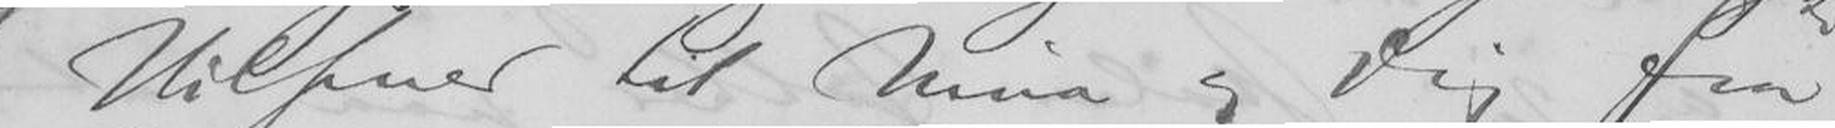Hilsener til Nina og Dig fra
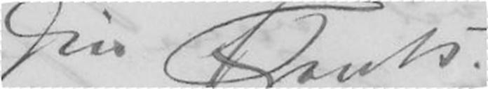Din Frants
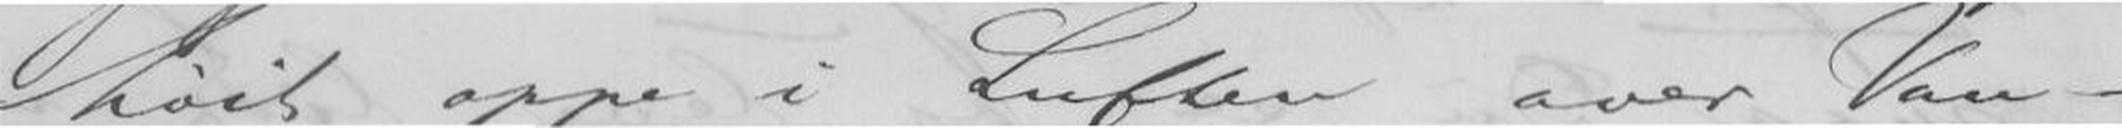høit oppe i Luften over Van-
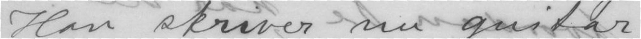Han skriver nu guitar-
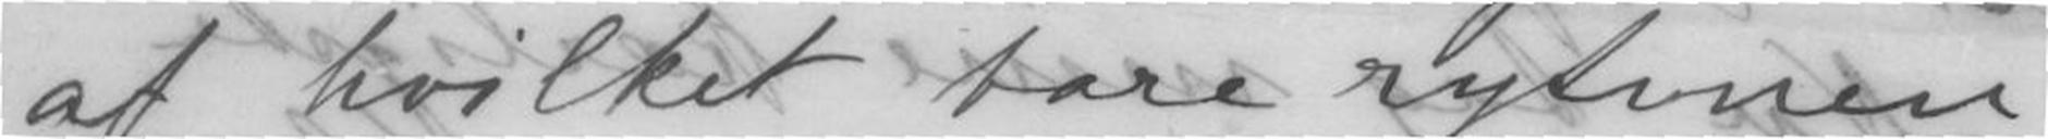af hvilket fare rytmen
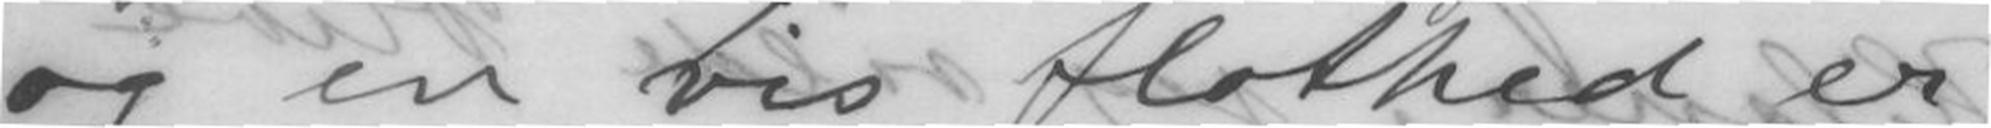og en vis flothed er
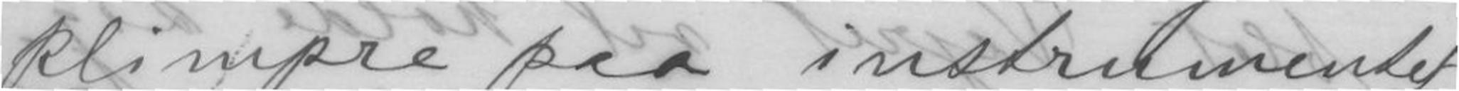klimpre paa instrumentet
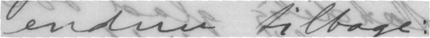endnu tilbage"
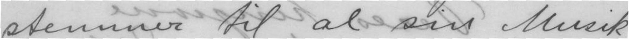stemmer til al sin Musik
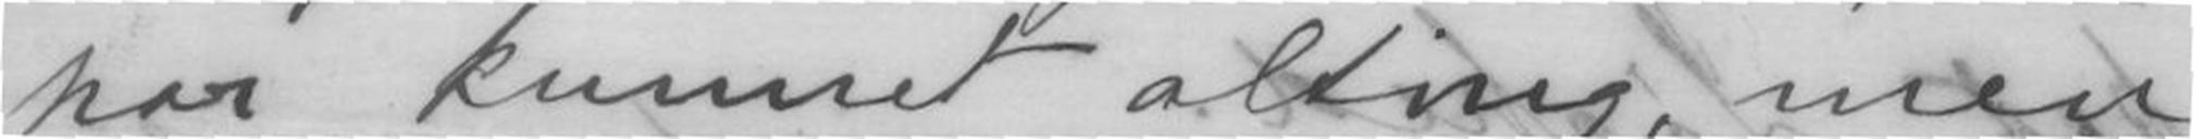har kunnet alting, men
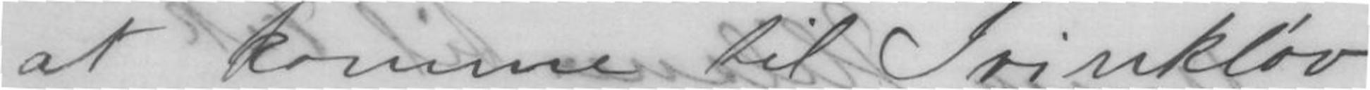at komme til Svinkløv
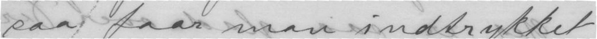saa faar man indtrykket
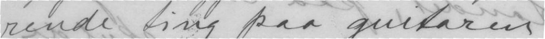rende ting paa guitaren;
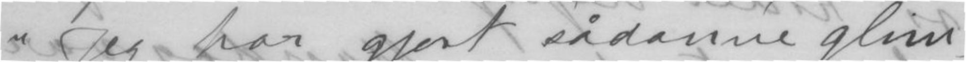"Jeg har gjort sadanne glim-
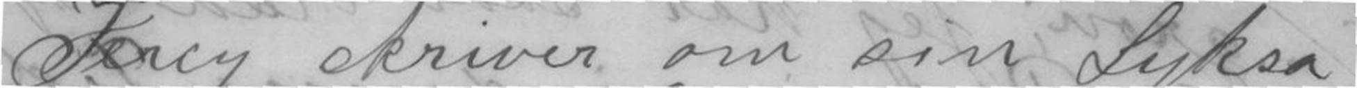Percy skriver om sin Lyksa-
